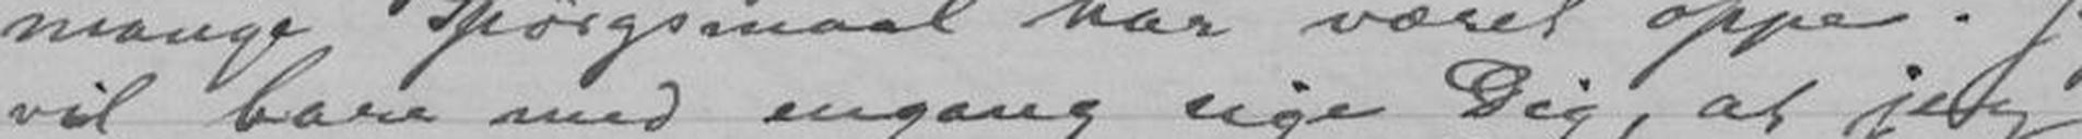vil bare med engang sige Dig, at jeg
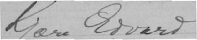Kjære Edvard!
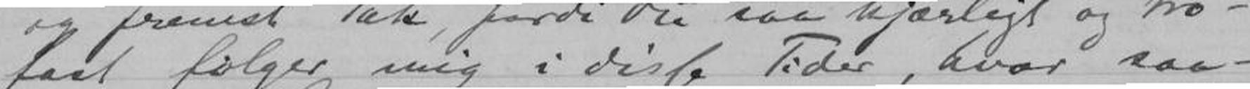fast følger mig i disse Tider, hvor saa-
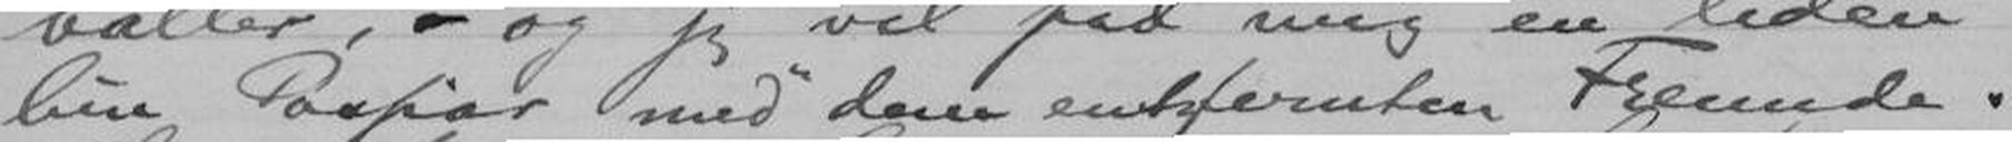lun Passiar med "dem entsfernten Freunde".
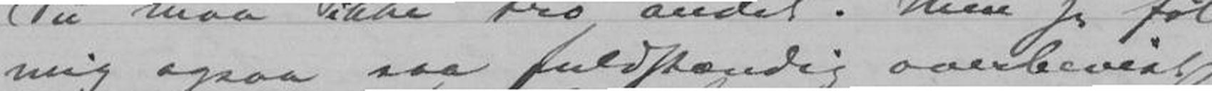mig ogsaa saa fuldstændig overbevist
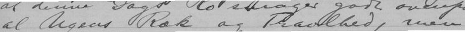al Ugens Ræk og Travelhed, men
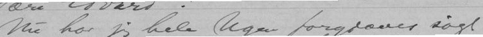Nu har jeg hele Ugen forgjæves søgt
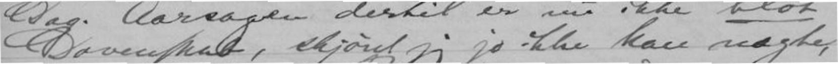Dovenskab, skjønt jeg jo ikke kan nægte,
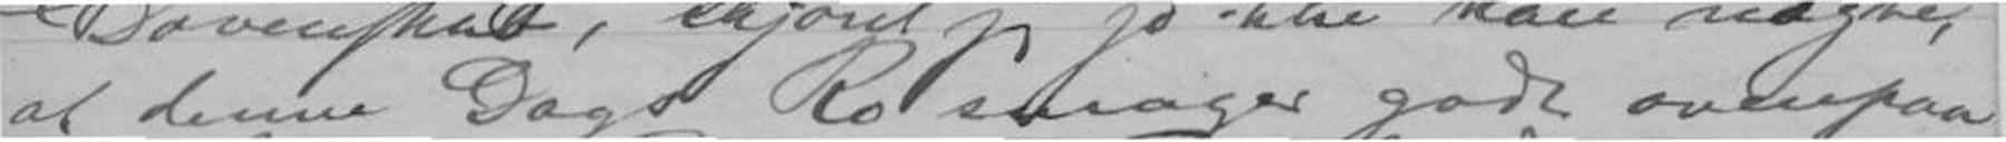at denne Dags Ro smager godt ovenpaa
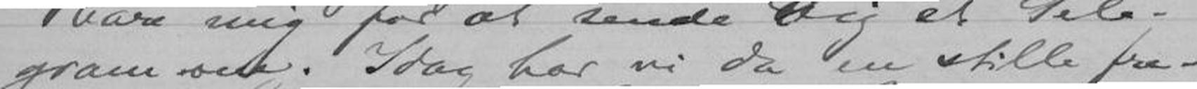gram om. Idag har vi da en stille fre-
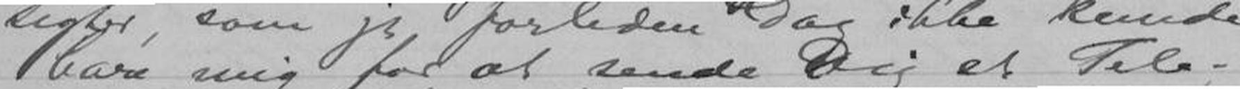bare mig for at sende Dig et Tele-
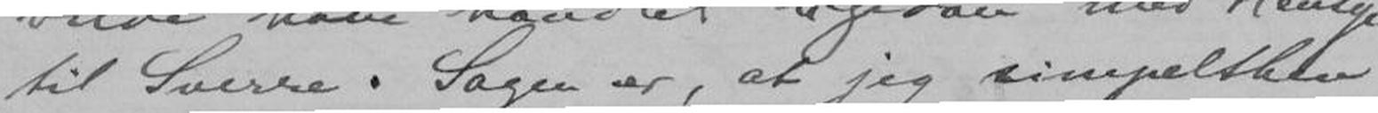til Sverre. Sagen er, at jeg simpelthen
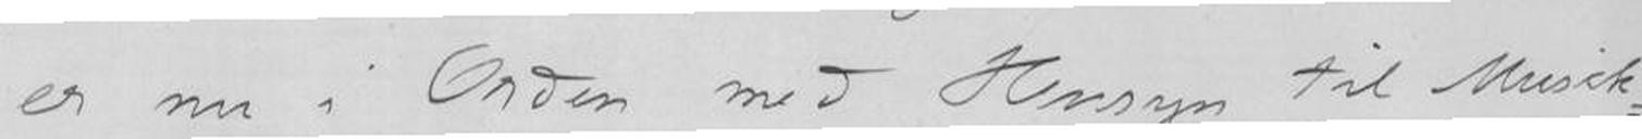er nu i Orden med Hensyn til Musik-
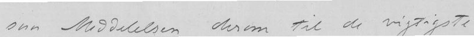saa meddelelsen derom til de vigtigste
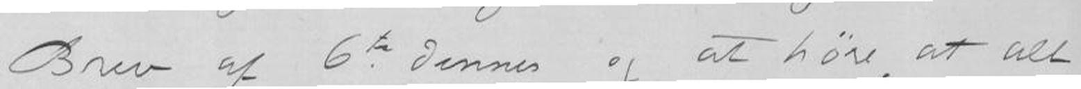Brev af 6te dennes og at høre, at alt
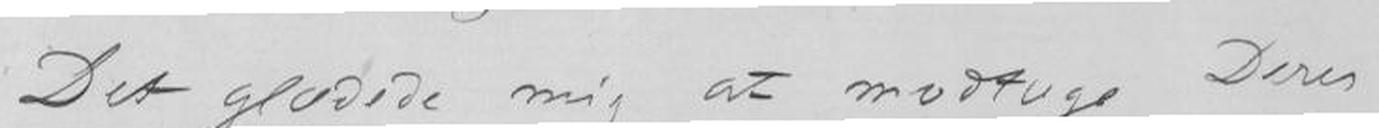Det glædede mig at modtage Deres
